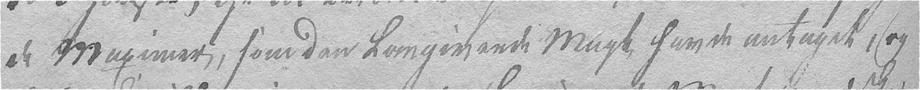de Maximer, som den Lovgivende Magt havde antaget, og
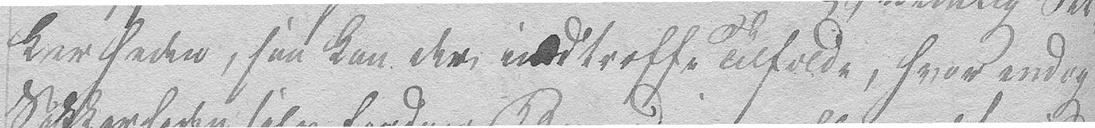kerheden, saa kan der indtræffe Tilfælde, hvor endog
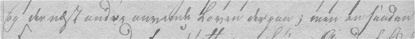og dernæst andre anvende Loven derpaa; men en saadan
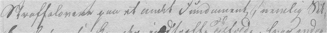Straffelovene paa et andet Fundament, nemlig Sik-
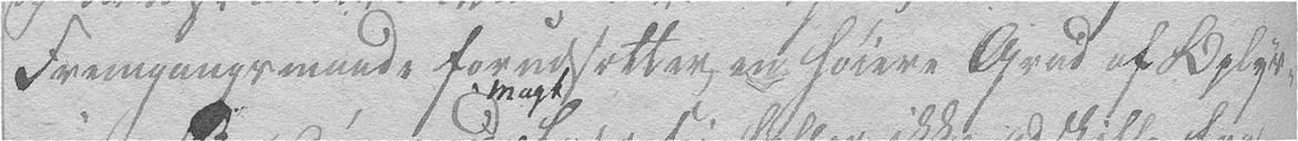Fremgangsmaade forudsætter en høiere Grad af Oplys-
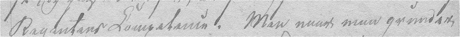Regentens Competence. Men naar man grunder
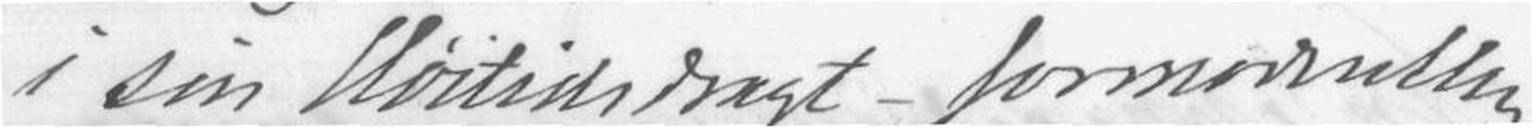i sin Høitidsdragt-formodentlig
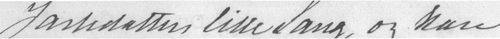Jarledatters lille Sang, og kan
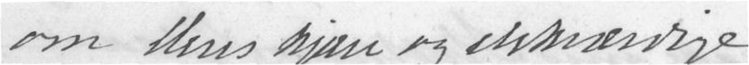om Deres kjære og elskværdige
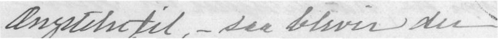Ængstelse til, - saa bliver der
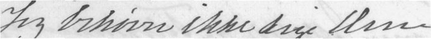Jeg behøver ikke sige Dem,
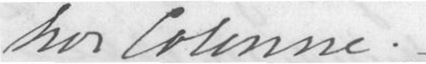hos Colonne. -
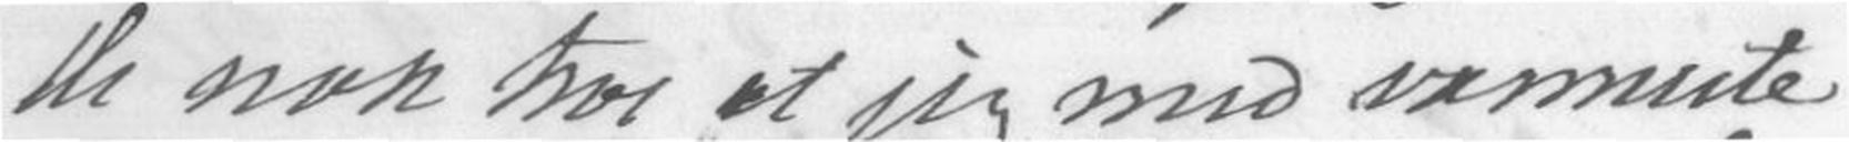De nok troe at jeg med varmeste
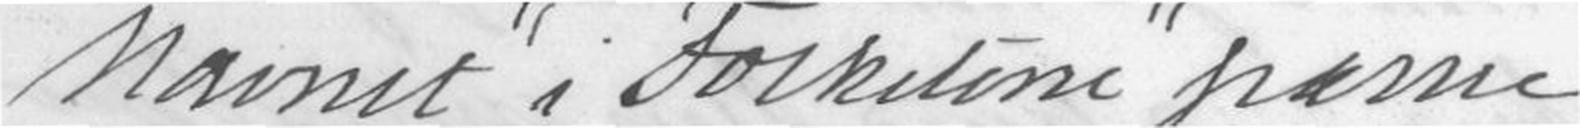Navnet "i Folketone" passer
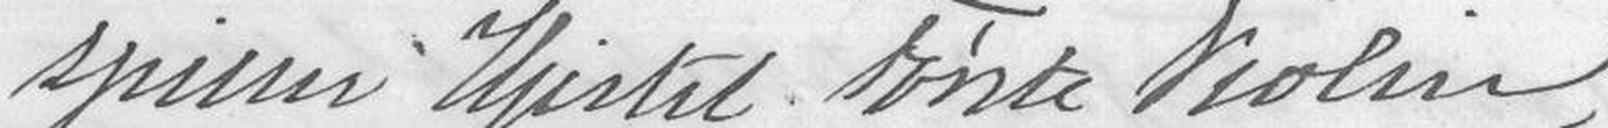spiller Hjertet Første Violin,
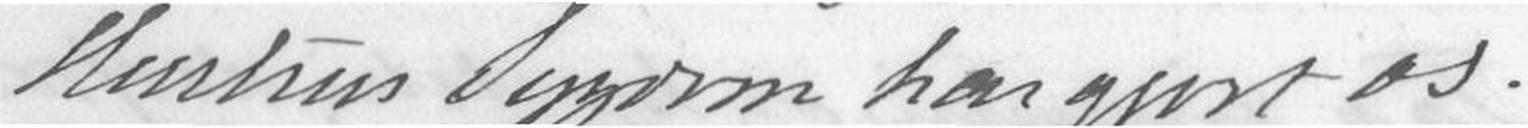Hustrus Sygdom har gjort os.
